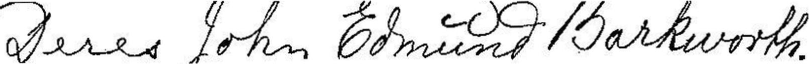Deres John Edmund Barkworth.
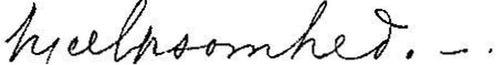hjelpsomhed. _
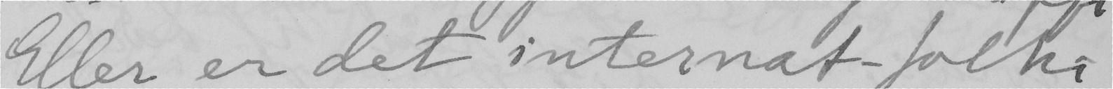Eller er det internat-folki
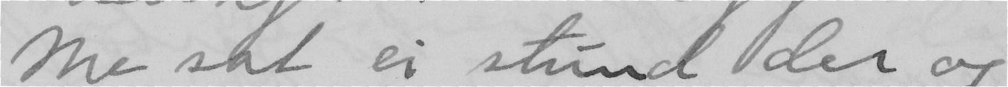Me sat ei stund der og
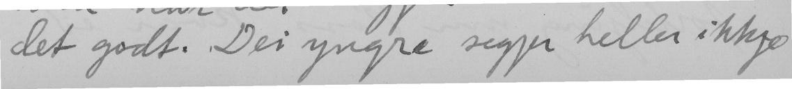det godt. Dei yngre segjer heller ikkje
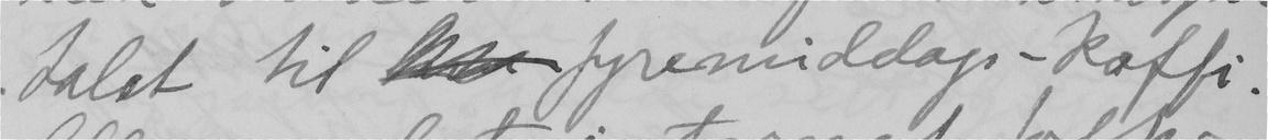talet til  fyremiddags-kaffi.
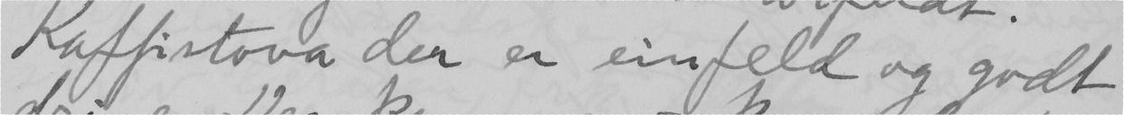Kaffistova der er einfeld og godt
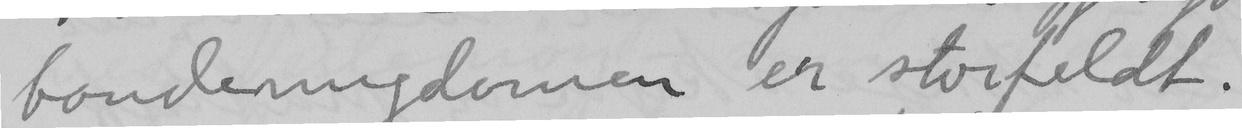bondeungdomen er storfeldt.
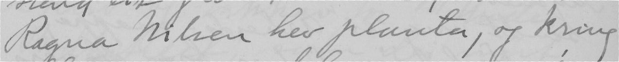Ragna Nilsen hev planta, og kring
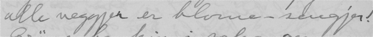alle veggjer er blome-sengjer.
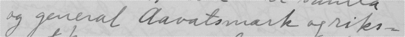og general Aavatsmark og riks-
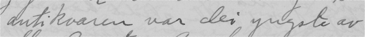antikvaren var dei yngste av
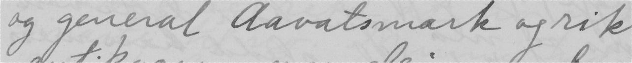tagal, likso byarkitekt Aars,
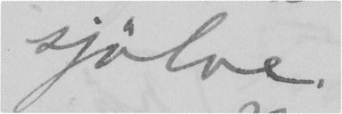sjölve.
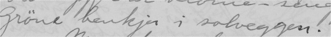Gröne benkjer i solveggen.
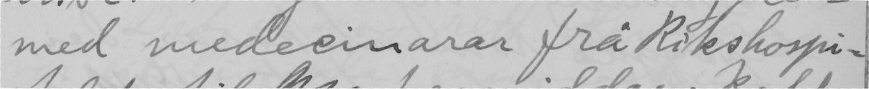med medeeinarar frå Rikshospi-
